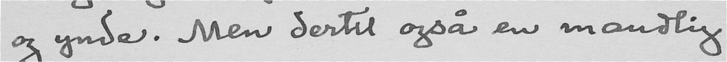og ynde. Men dertil også en mandlig
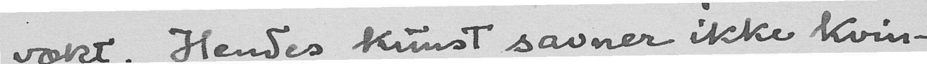vækt. Hendes kunst savner ikke kvin-
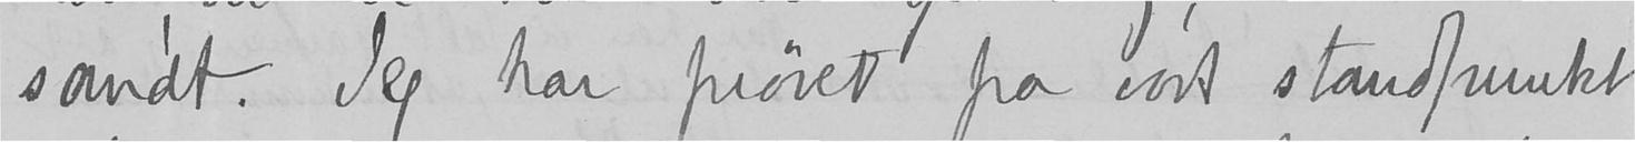sandt. Jeg har prøvet fra vort standpunkt
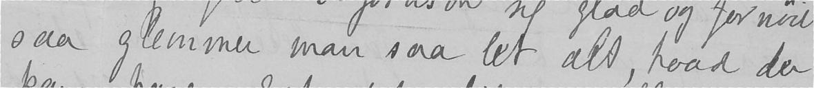saa glemmer man saa let, alt hvad der
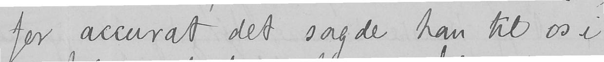for accurat det sagde han til os i
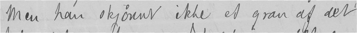Men han skjønner ikke et gran af det
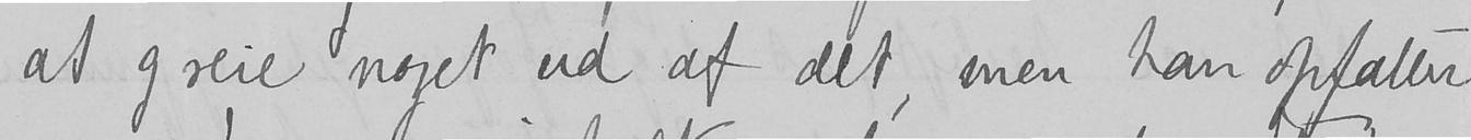at greie noget ud af det, men han opfatter
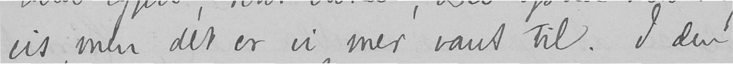vis, men det er vi mer vant til. I den
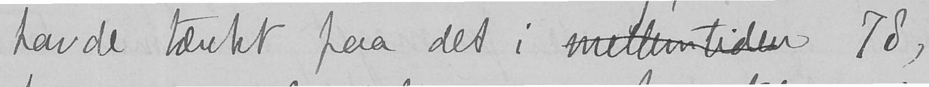havde tænkt paa det i mellemtiden 78,
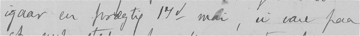igaar en prægtig 17d mai, vi vare paa
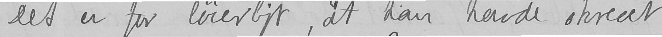Det er for løierligt, at han havde skrevet
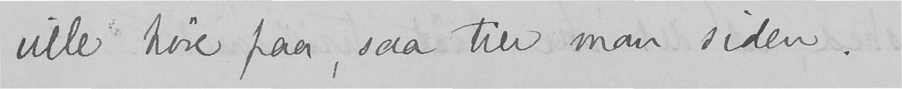ville høre paa, saa tier man siden.
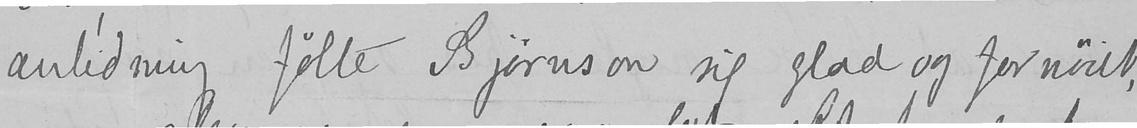anledning følte Bjørnson sig glad og fornøiet,
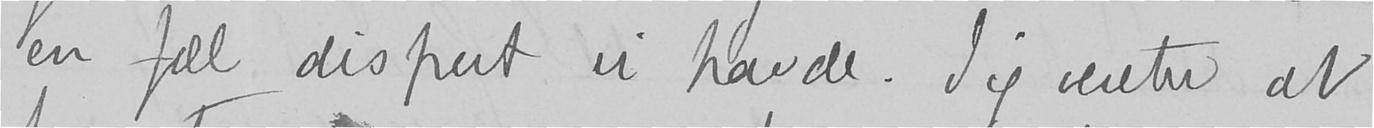en fæl disput vi havde. Jeg venter at
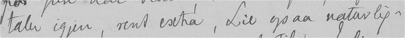taler igjen, rent extra, Lie ogsaa naturlig-
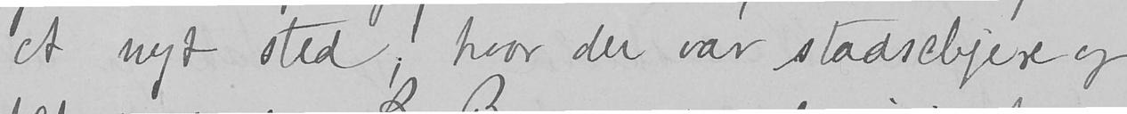et nyt sted, hvor der var stadseligere og
